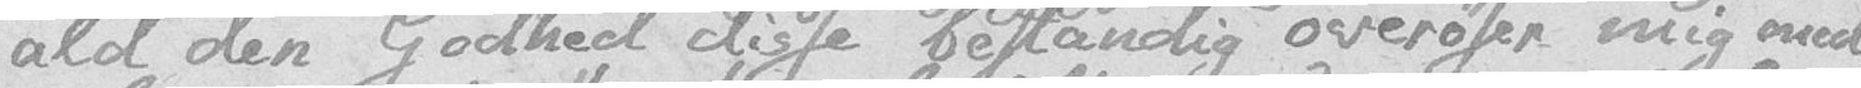ald den Godhed disse bestandig overøser mig med
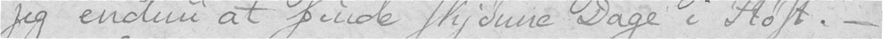jeg endnu at finde skjønne Dage i Høst. –
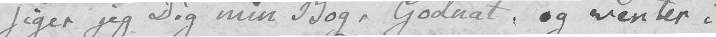siger jeg Dig min Bog, Godnat, og venter i
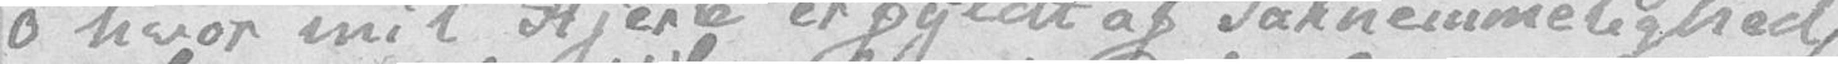o hvor mit Hjerte er fyldt af Taknemmelighed,
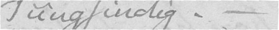Tungsindig. –
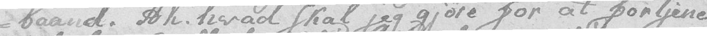-baand. Ak! hvad skal jeg gjøre for at fortjene
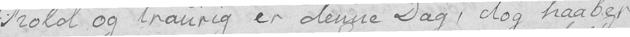Kold og traurig er denne Dag, dog haaber
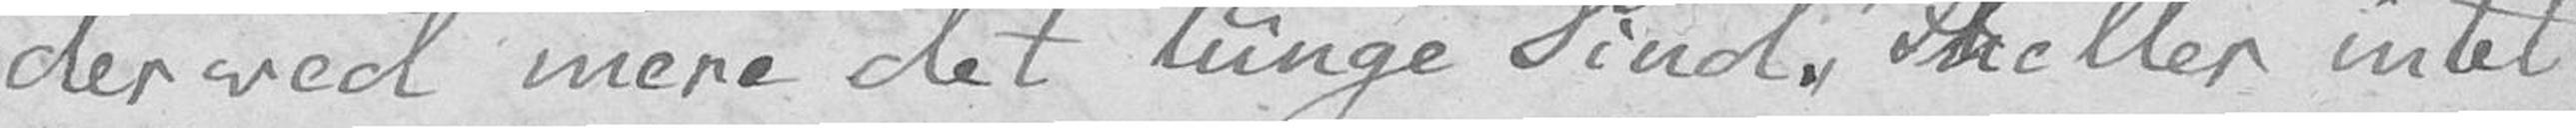derved mere det tunge Sind. Heller intet
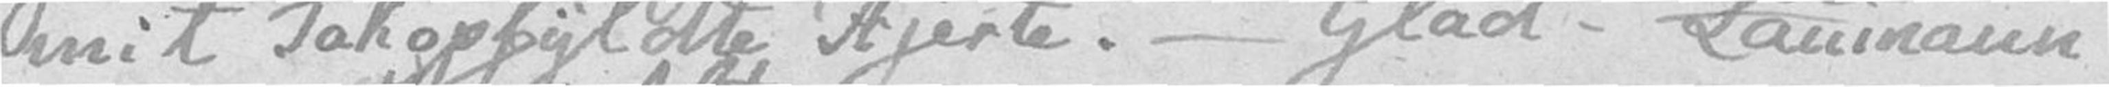mit Takoppfyldte Hjerte. – Glad – Laumann
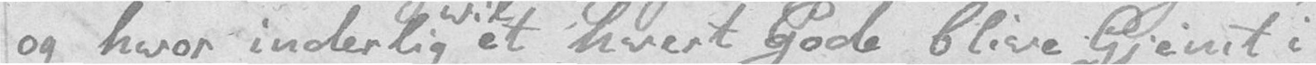og hvor inderlig vil et hvert Gode blive Gjemt i
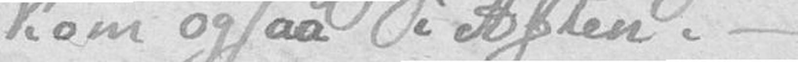kom ogsaa i Aften. –
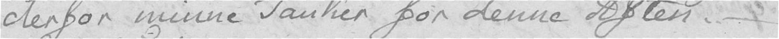derfor minne Tanker for denne Aften. –
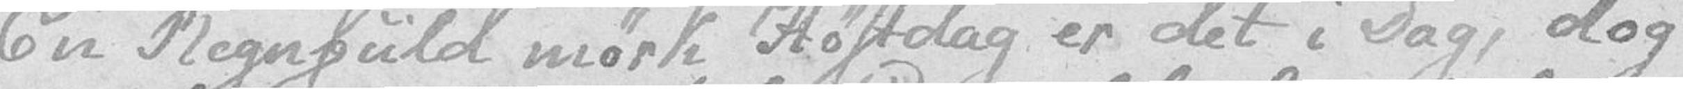En Regnfuld mørk Høstdag er det i Dag, dog
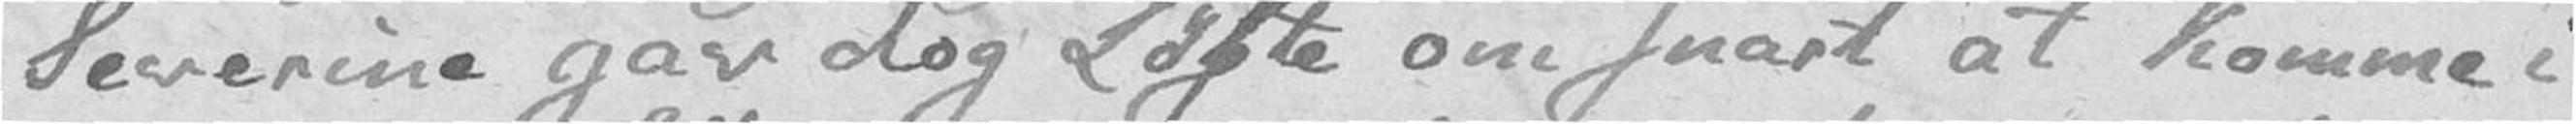Severine gav dog Løfte om snart at komme i
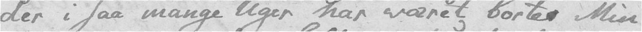der i saa mange Uger har været borte. Min
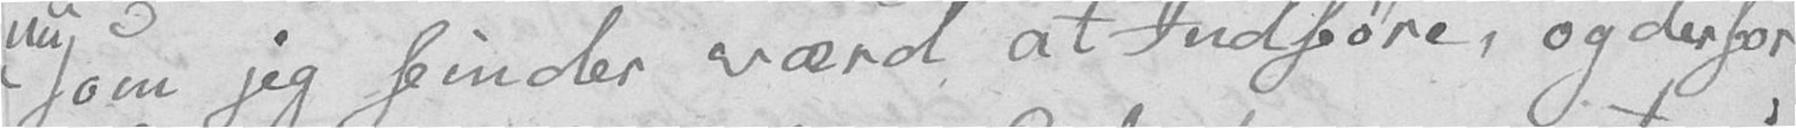nu som jeg finder værd at Indføre, og derfor
